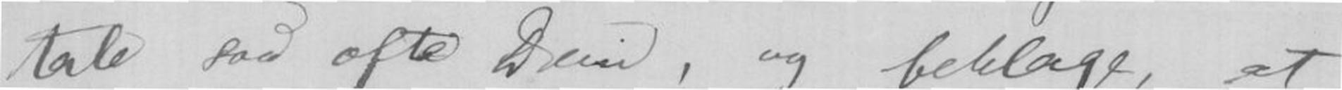tale saa ofte Dem, og beklage, at
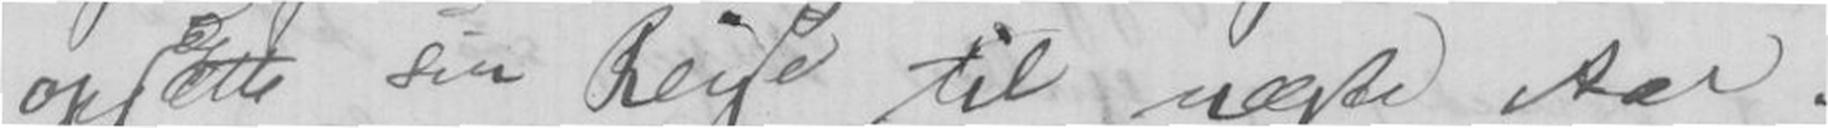opsætte sin Reise til næste Aar.
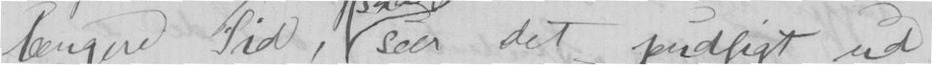længere Tid, saa seer det pudsigt ud,
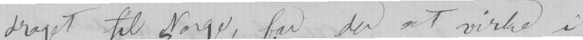draget til Norge, for der at virke i
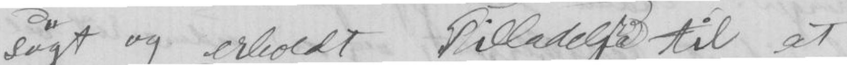søgt og erholdt Tilladelse til at
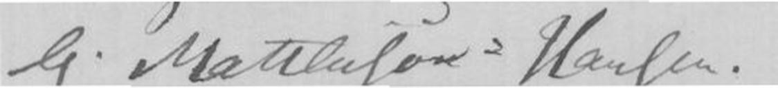G. Matthison-Hansen
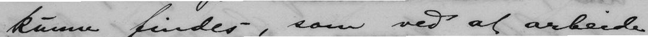kunne findes, som ved at arbeide
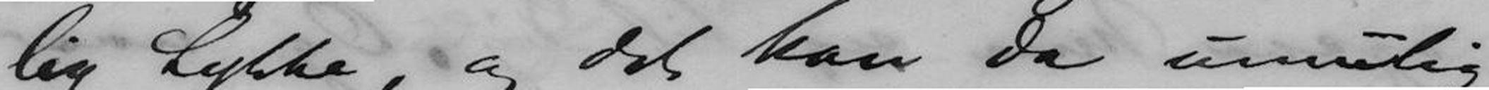lig Lykke, og det kan da umulig
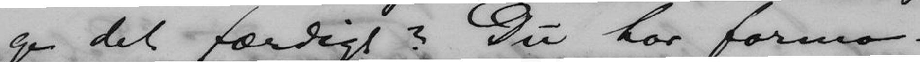ge det færdigt? Du har formo-
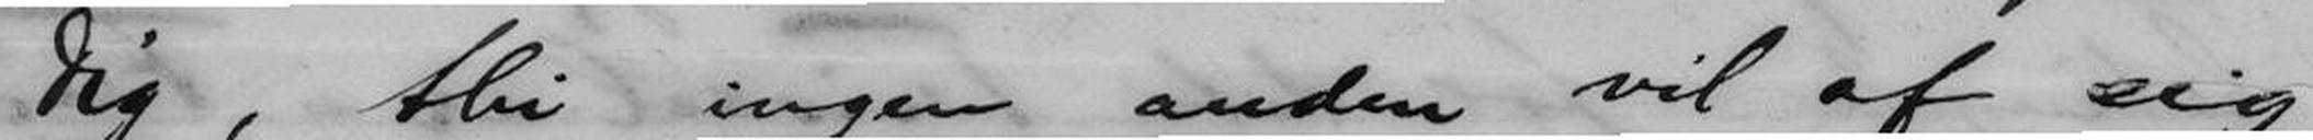Dig, thi ingen anden vil af sig
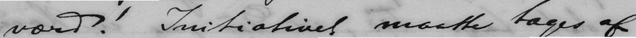værd! Initiativet maatte tages af
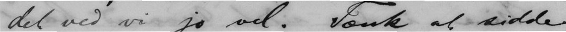det ved vi jo vel. Tænk at sidde
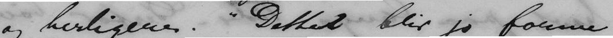og herligere. Dette blir jo forme-
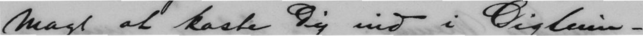Magt at kaste Dig ind i Digtnin-
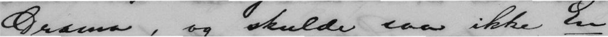Drama, og skulde saa ikke En
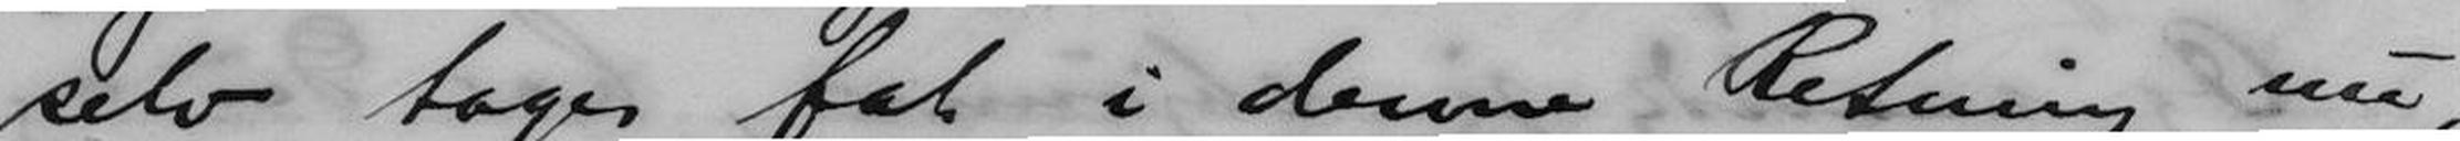selv tage fat i denne -Retning nu,
